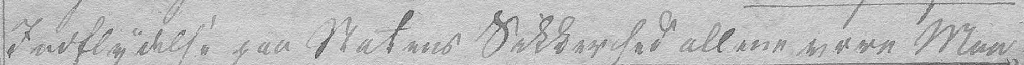Indflydelse paa Statens Sikkerhed allene være Maa-
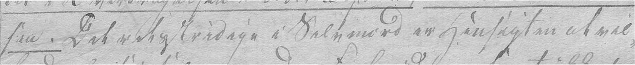sen. Det retsstridige i Selvmord er Hensigten at vil-
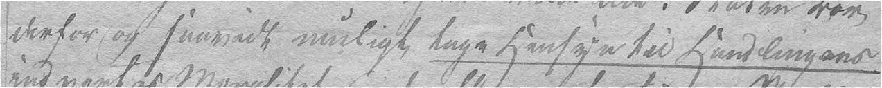derfor og saavidt muligt tage Hensyn til Handlingens
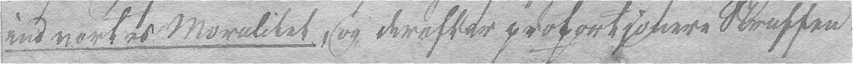indvortes Moralitet, og derefter proportionere Straffen.
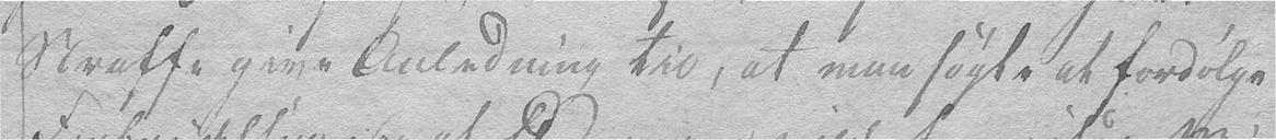Straffe give Anledning til, at man søgte at fordølge
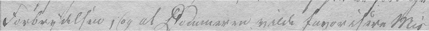Forbrydelsen, og at Dommeren vilde favorisere Mis-
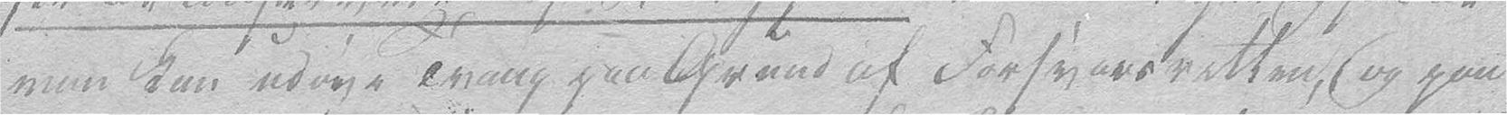man kan udøve Tvang paa Grund af Forsvarsretten, og paa-
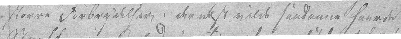større Forbrydelser, dernæst vilde saadanne haarde
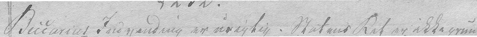Beccarias Indvending er urigtig. Statens Ret er ikke grun-
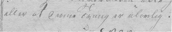eller at denne Tvang er ulovlig.
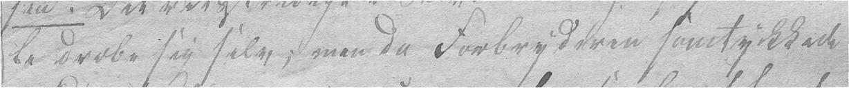le dræbe sig selv, men da Forbryderen samtykkede
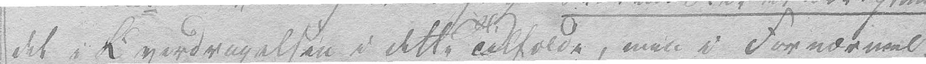det i Overdragelsen i dette Tilfælde, men i Fornærmel-
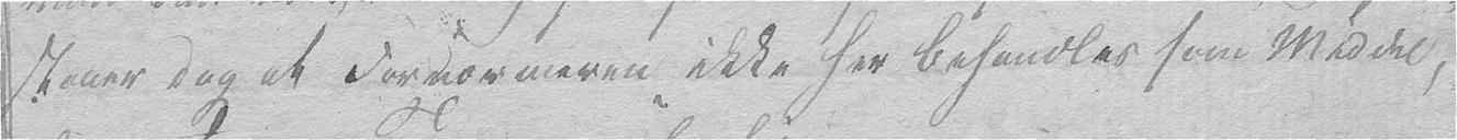staaer dog at Fornærmeren ikke her behandles som Middel,
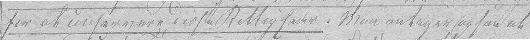for at conservere disse Rettigheder. Man antager ogsaa at
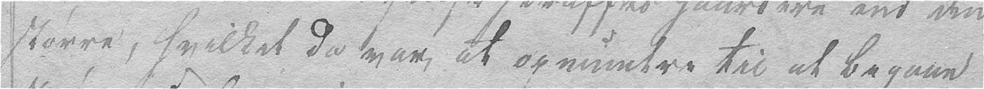større, hvilket da var at opmuntre til at begaae
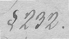§ 232.
